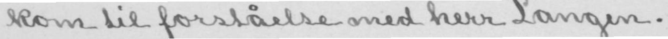kom til forståelse med herr Langen.
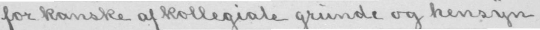for kanske af kollegiale grunde og hensyn
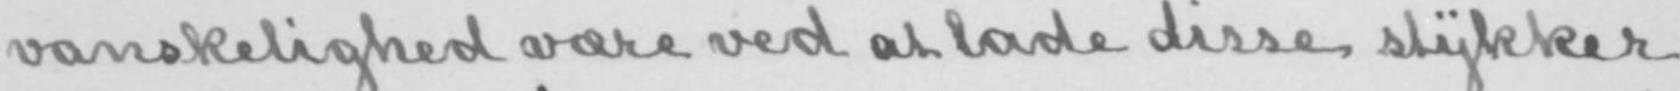vanskelighed være ved at lade disse stykker
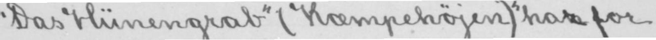«Das Hünengrab» (Kæmpehøjen)» hanr for
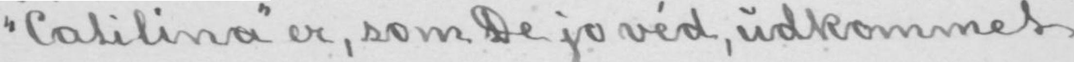«Catilina» er, som De jo véd, udkommet
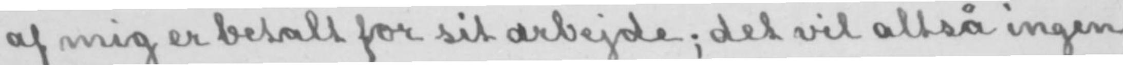af mig er betalt for sit arbejde; det vil altså ingen
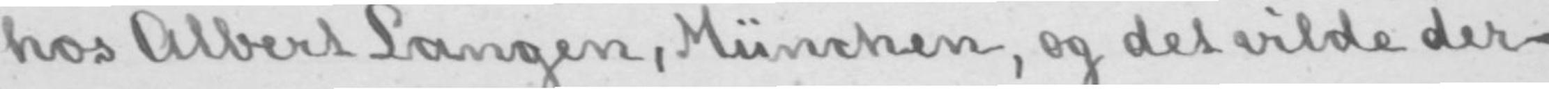hos Albert Langen, München, og det vilde der-
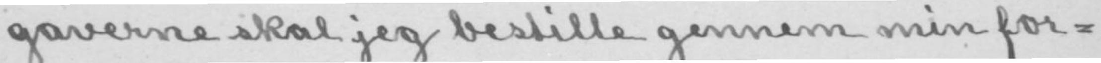gaverne skal jeg bestille gennem min for-
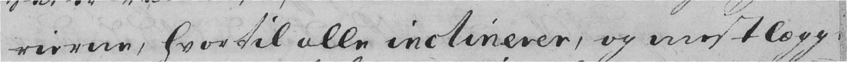rierne, hvortil alle inclinerer, og mest lægg
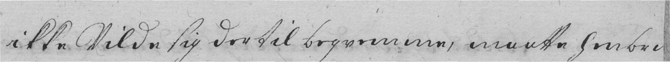ikke Vilde sig dertil beqvemme, maatte henbrin-
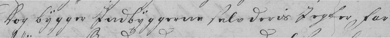Dog bygger Indbyggerne selv deres Iegter Far-
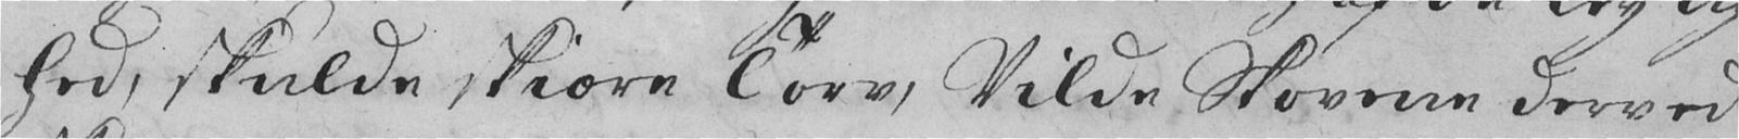hed, skulde skiære Torv, vilde Skovene derved
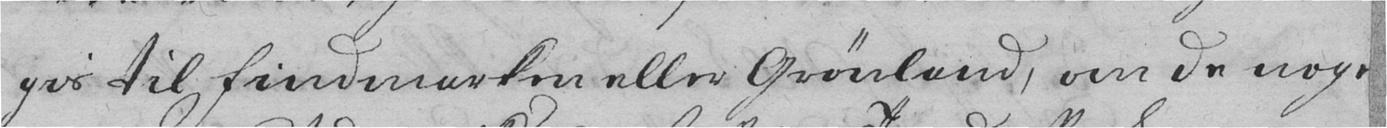gis til Findmarken eller Grønland, om de nogen
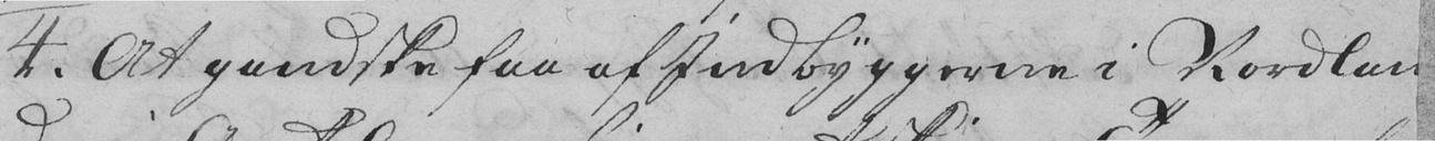4. At gandske faa af Indbyggerne i Nordlan-
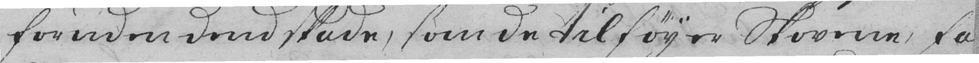foruden dend skade, som de tilføyer Skovene, fa
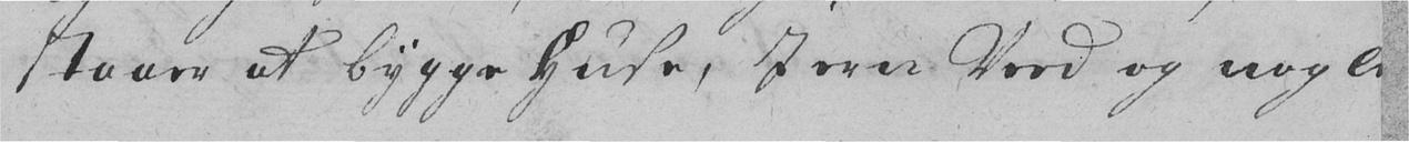staaer at bygge Huse, Iern Veed og nogle
